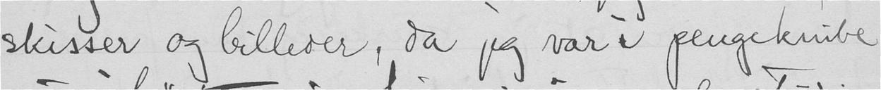skisser og billeder, da jeg var i pengeknibe
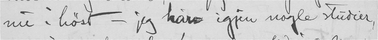nu i høst - jeg har igjen nogle studier,
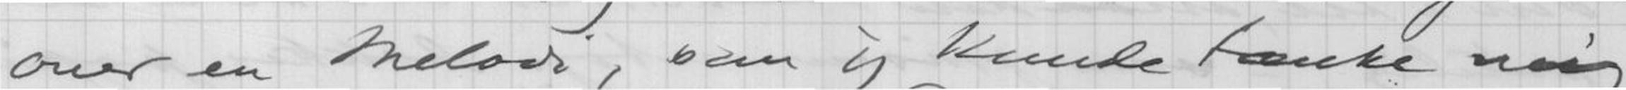over en melodi, som jeg kunde tænke mig
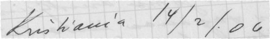Kristiania 14/2/06
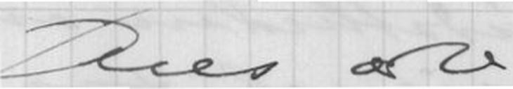Deres ære
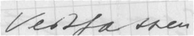Vestfasjen
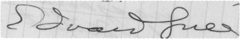Edvard Grieg
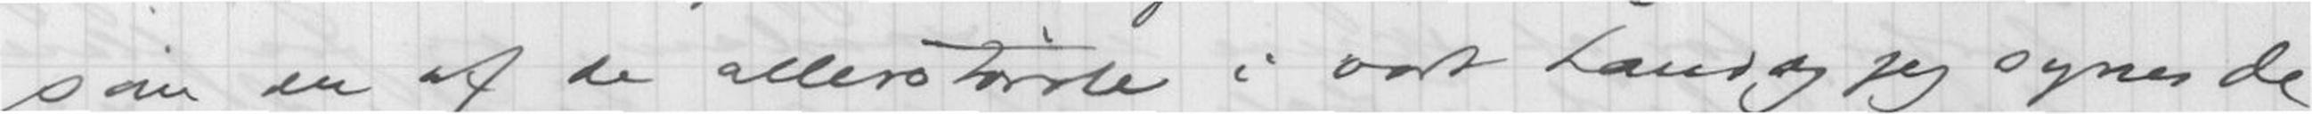som en af de aller største i vort land og jeg synes de
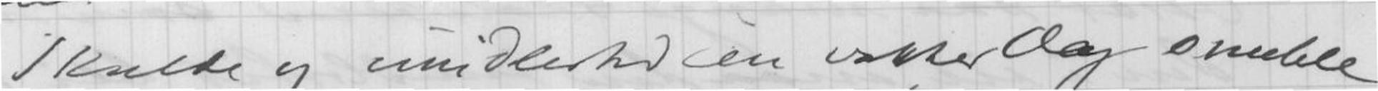Skulde jeg imidlertid en vakker dag snuble
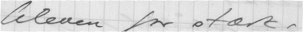bleven for stærk.
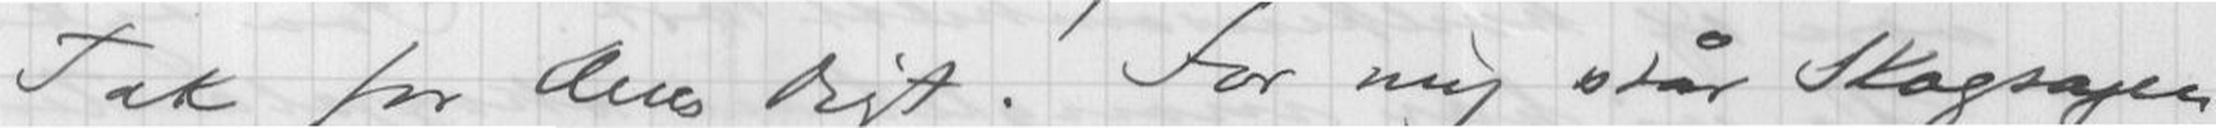Tak for Deres digt! For mig står Skogsagen
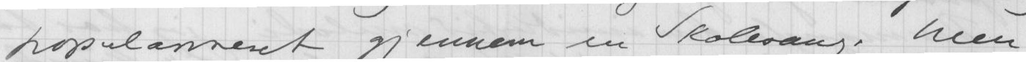populære rent gjennem en Skolesang. Men
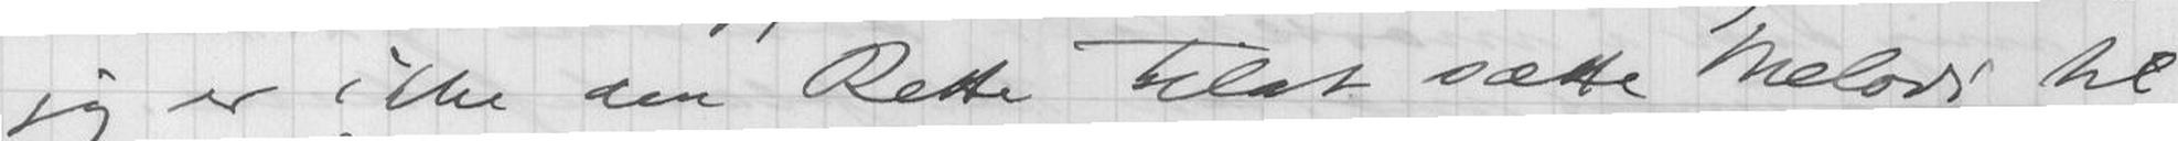jeg er ikke den Rette til at sætte melodi til
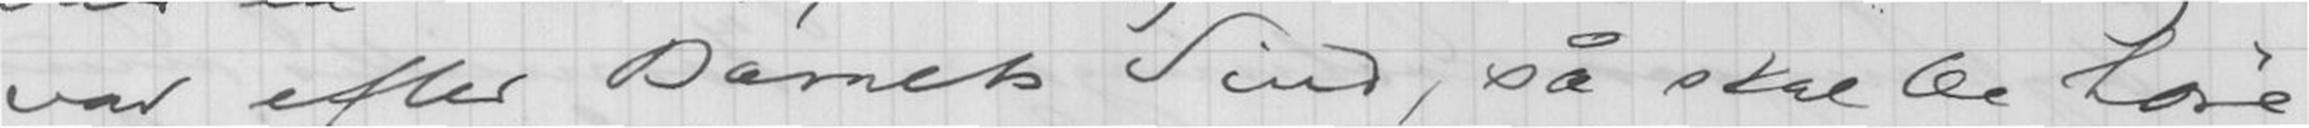var efter Dæmets Sind, så skal De høre
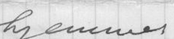hjemmes
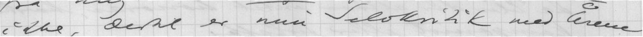ikke, der til er nuu Selvkritiken med
